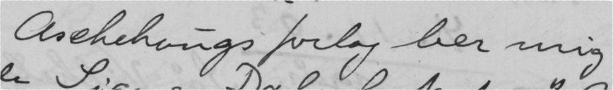Aschehougs forlag ber mig
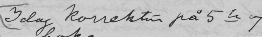Idag korrektur på 5te og
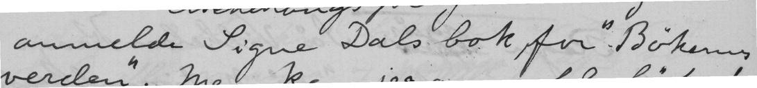anmelde Signe Dals bok for "Bøkenes
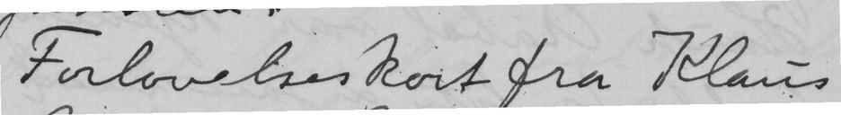Forlovelseskort fra Klaus
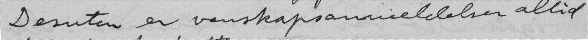Desuten er venskapsanmeldelser altid
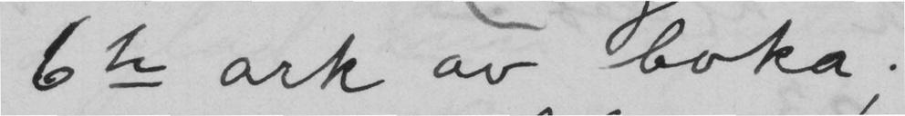6te ark av boka.
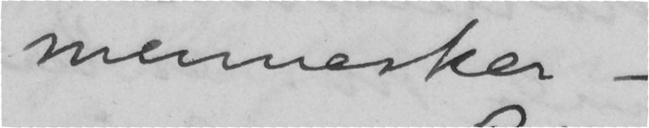mennesker -
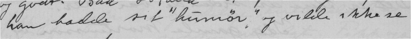han hadde sit "humør" og vilde ikke se
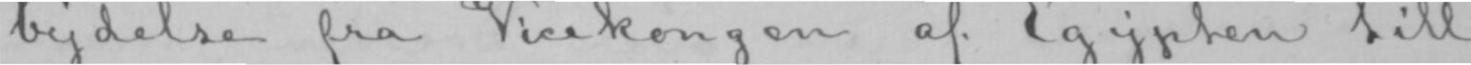bydelse fra Vicekongen af Egypten till
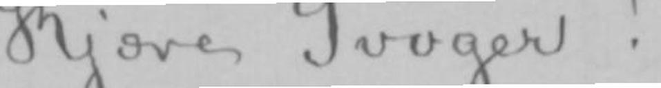Kjære Svoger!
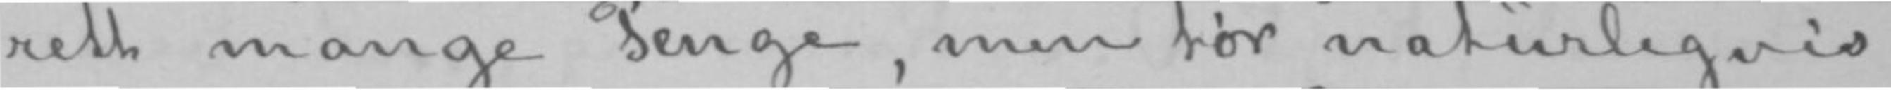rett mange Penge, men tør naturligvis
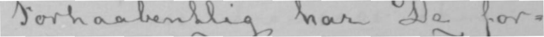Forhaabentlig har De for-
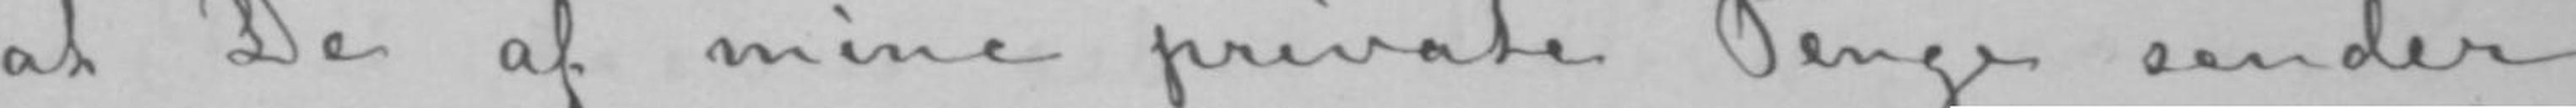at De af mine private Penge sender
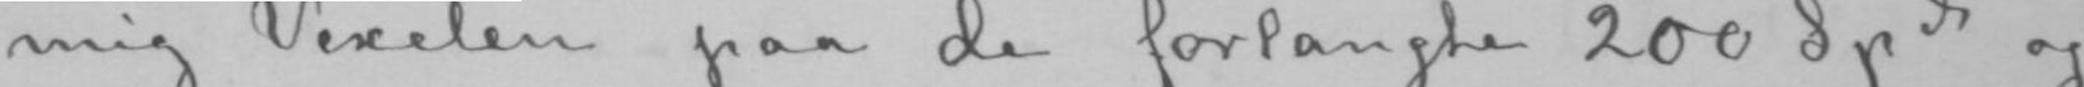mig Vexelen paa de forlangte 200 Spd og
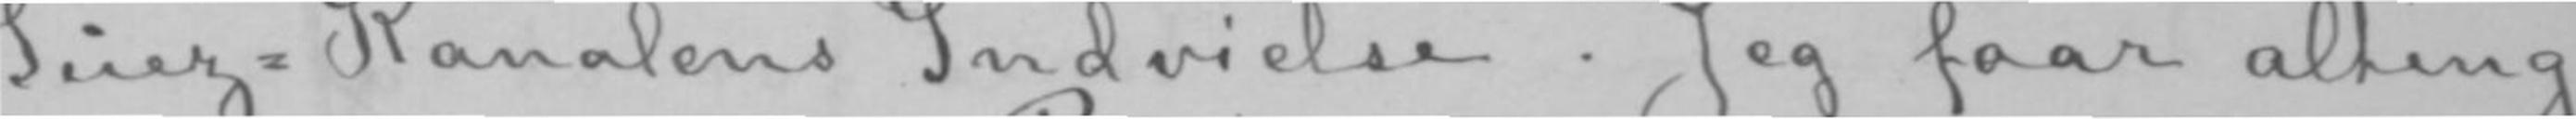Suez-Kanalens Indvielse. Jeg faar alting
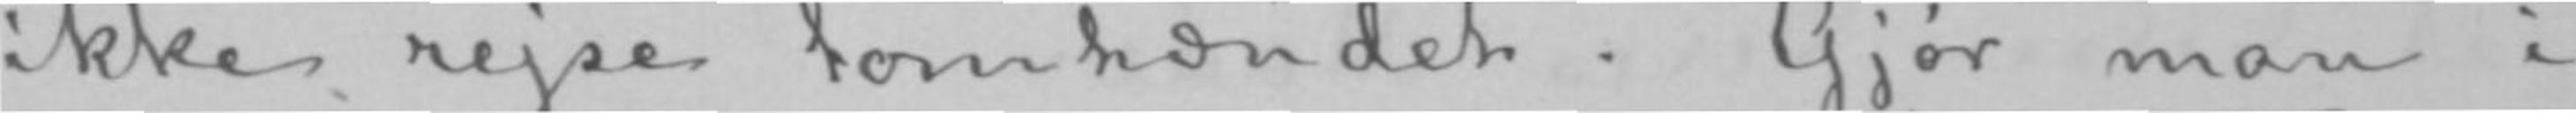ikke rejse tomhændet. Gjør man i
